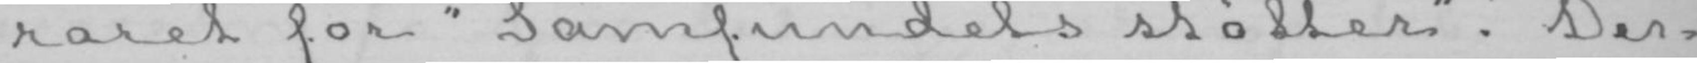raret for «Samfundets støtter». Der-
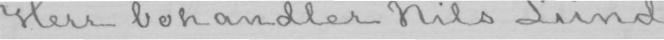Herr bohandler Nils Lund.
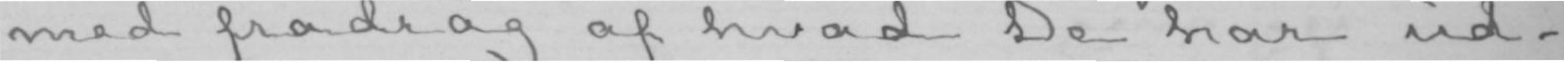med fradrag af hvad De har ud-
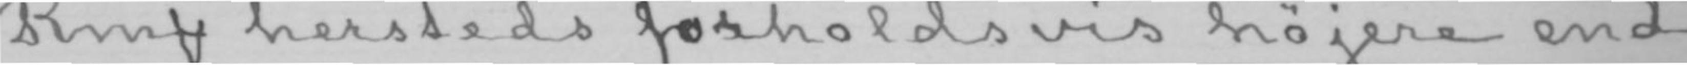RmՖ hersteds forholdsvis højere end
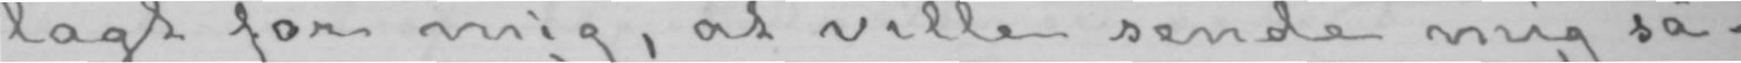lagt for mig, at ville sende mig så-
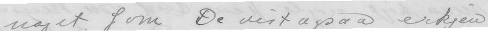noget, som De vist ogsaa erkjen-
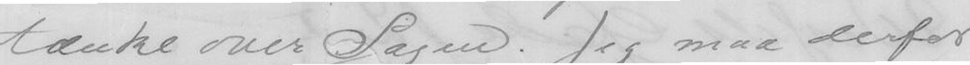tænke over Sagen. Jeg maa derfor
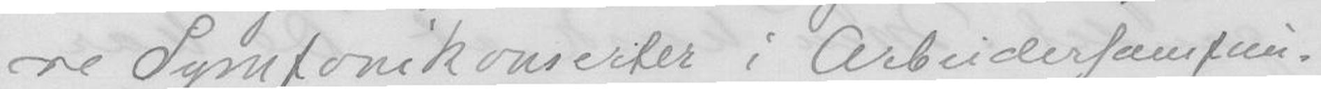re Symfonikonserter i Arbeiderforfun-
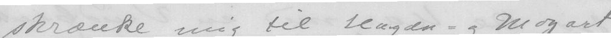skrænke mig til Haydn= og Mozart
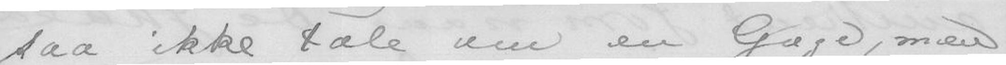saa ikke tale om en Gage, men
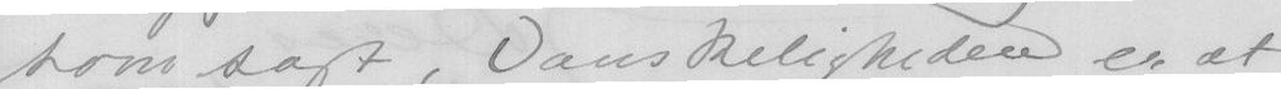som sagt, Vanskeligheden er at
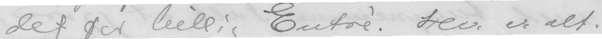det for billig Entré. Her er alt-
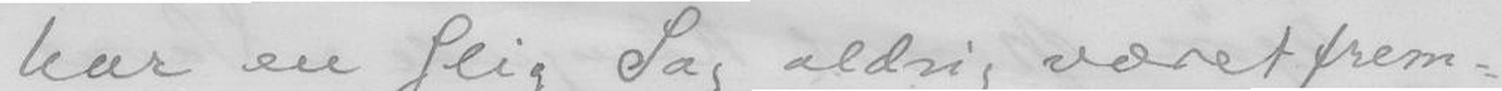har en slig Sag aldrig været frem-
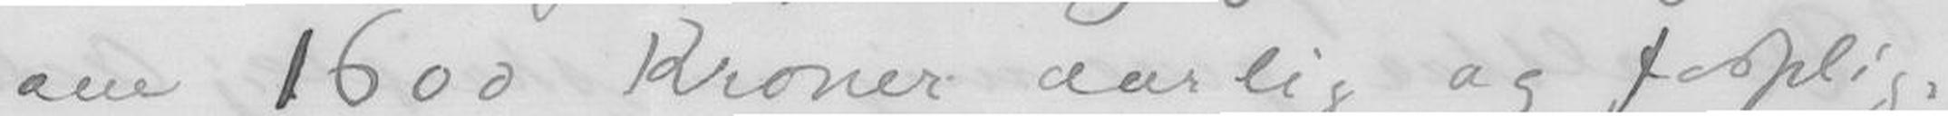om 1600 Kroner aarlig og forplig-
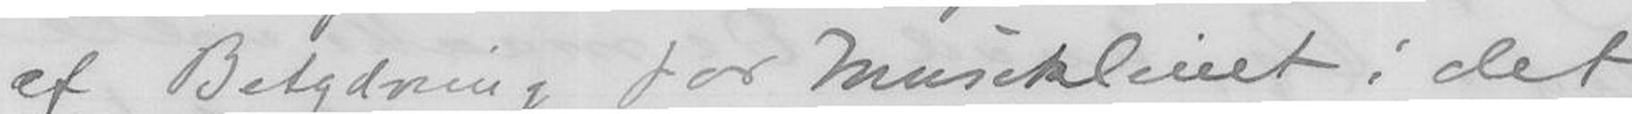af Betydning for Musiklivet i det
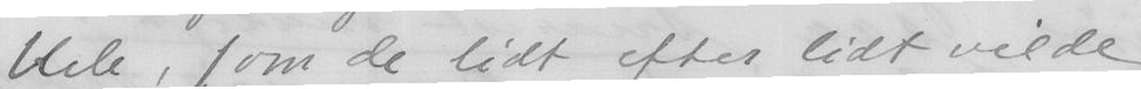Hele, som de lidt efter lidt vilde
